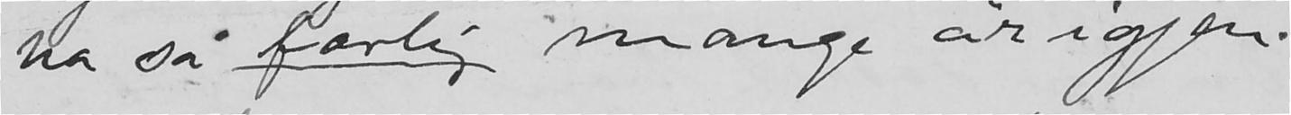ha så farlig mange år igjen.
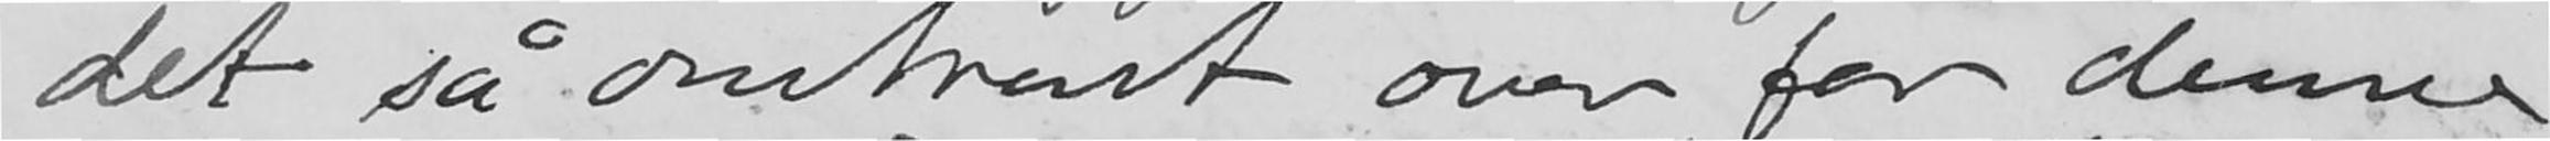det så omtrent over for denne
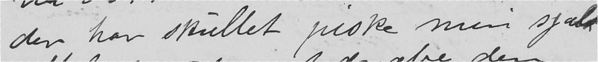der har skullet piske min sjæl
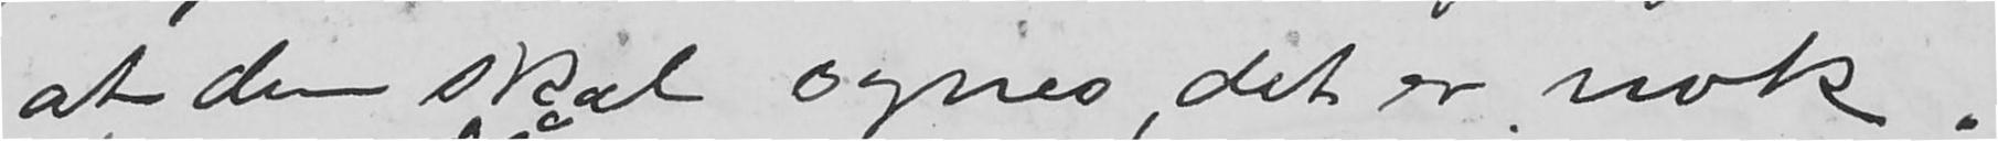at den skal synes, det er nok.
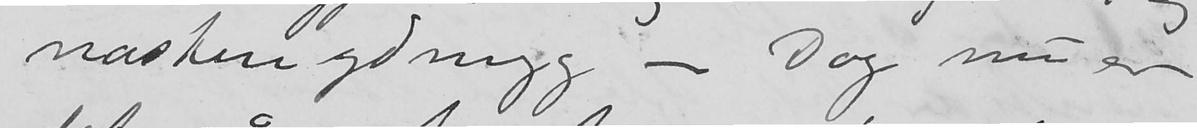næsten ydmyg - Dog nu er
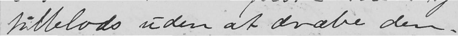tilblods uden at dræbe den.
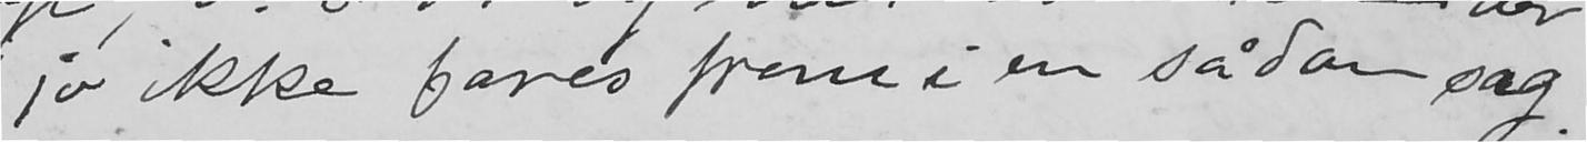jo ikke fares frem i en sådan sag.
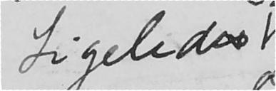Ligeledes
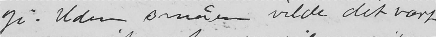gi. Uden småen vilde det vært
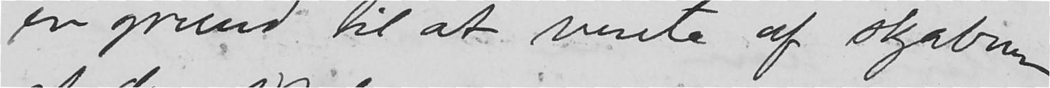er grund til at vente af skæbnen
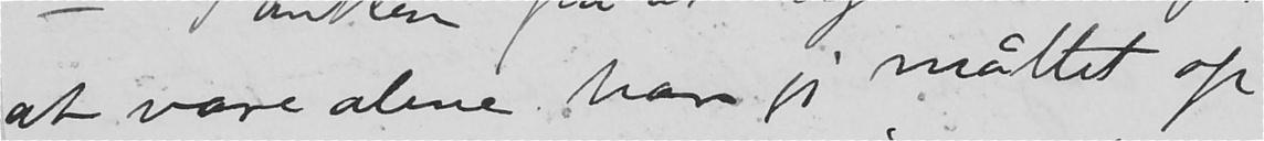at være alene har jeg måttet op
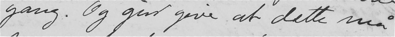gang. Og gud give at dette må
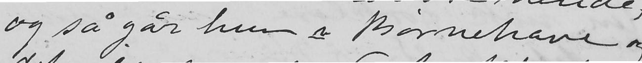og så går hun i Børnehave og
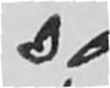så
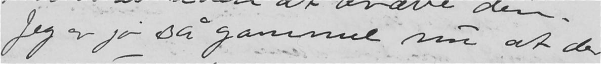Jeg er jo så gammel nu at der
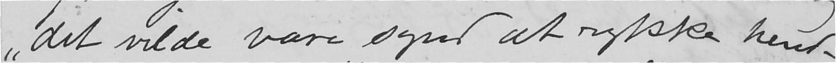"det vilde være synd at rykke hende
